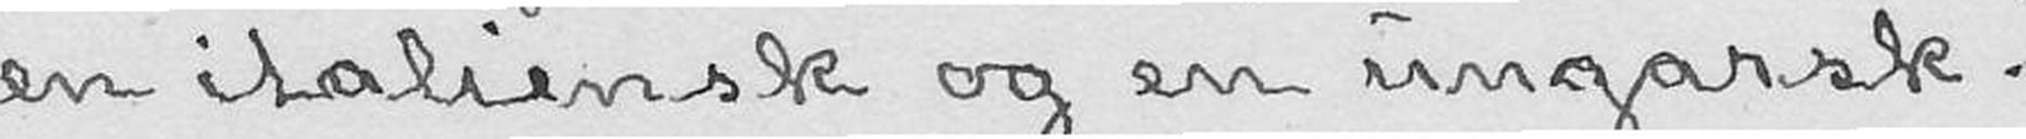en italiensk og en ungarsk.
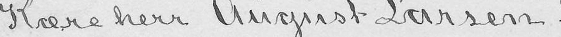Kære herr August Larsen!
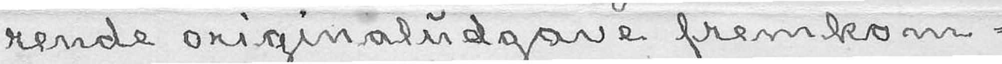rende originaludgave fremkom-
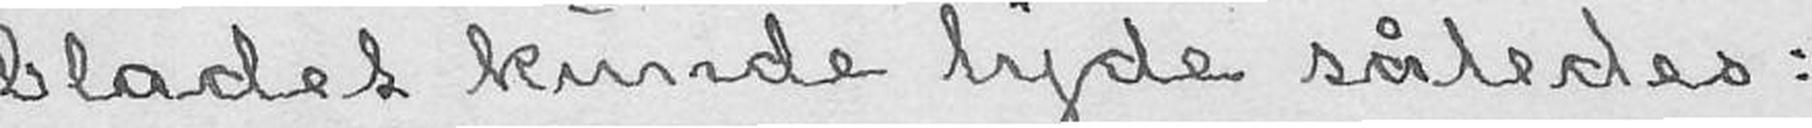bladet kunde lyde således:
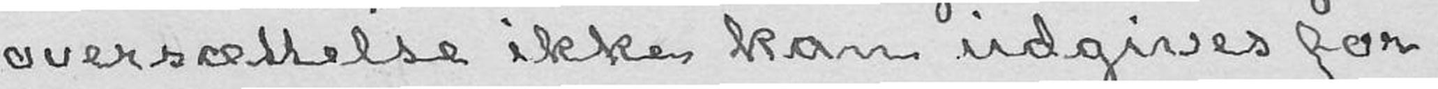oversættelse ikke kan udgives for-
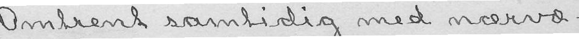Omtrent samtidig med nærvæ-
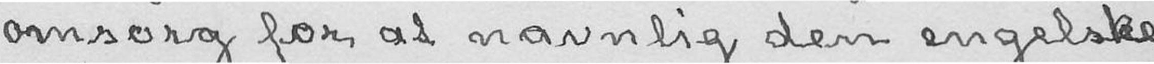omsorg for at navnlig den engelseke
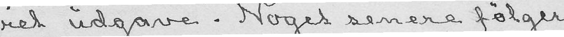ret udgave. Noget senere følger
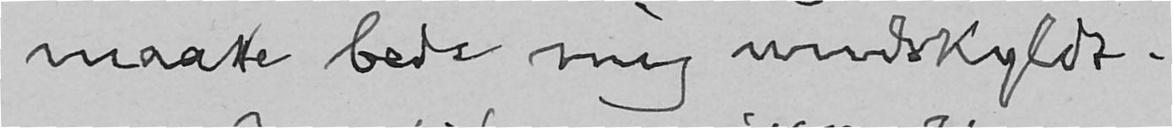maatte bede mig undskyldt.
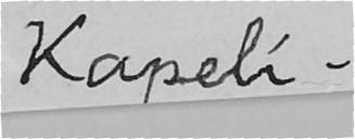Kapeli.
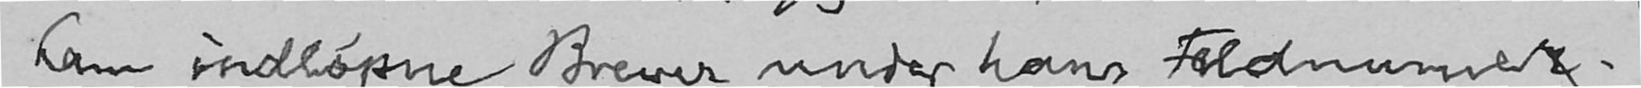ham indløpne Brever under hans Feldnumrer.
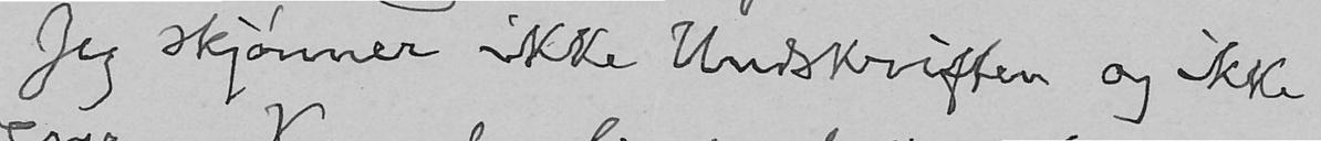Jeg skjønner ikke Undskriften og ikke
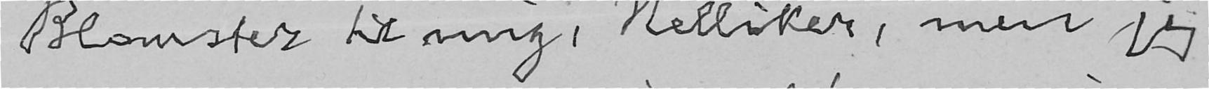Blomster til mig, Nelliker, men jeg
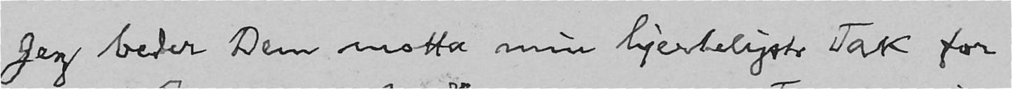Jeg beder Dem motta min hjerteligste Tak for
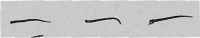- - -
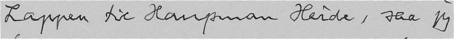Lappen til Haupman Heide, saa jeg
